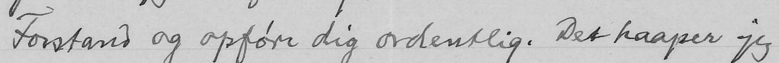Forstand og opføre dig ordentlig. Det haaper jeg
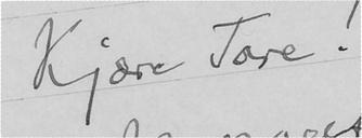Kjære Tore!
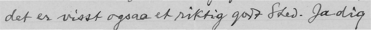det er visst ogsaa et riktig godt Sted. Ja dig
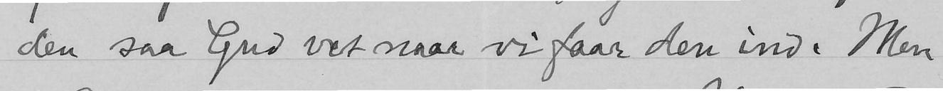den saa Gud vet naar vi faar den ind. Men
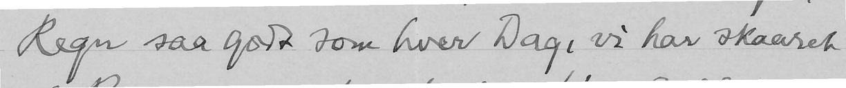Regn saa godt som hver Dag, vi har skaaret
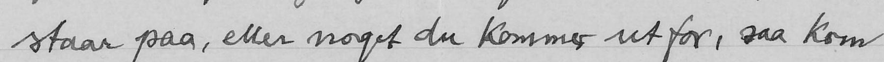staar paa, eller noget du kommer ut for, saa kom
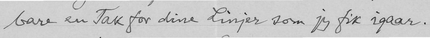bare en Tak for dine Linjer som jeg fik igaar.
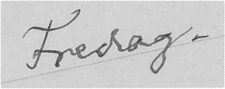Fredag.
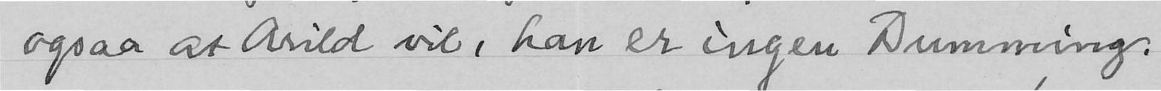ogsaa at Arild vil, han er ingen Dumming.
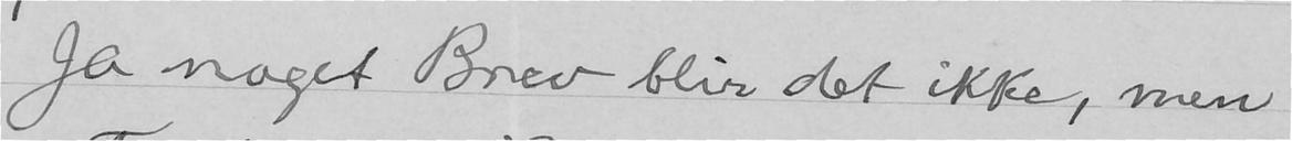Ja noget Brev blir det ikke, men
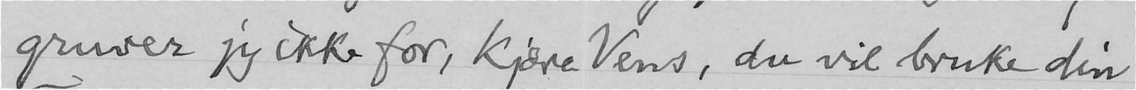gruver jeg ikke for, kjære Vens, du vil bruke din
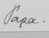Papa.
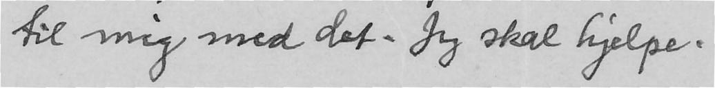til mig med det. Jeg skal hjelpe.
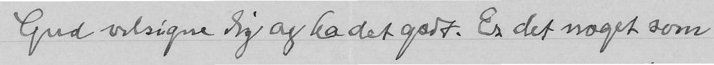Gud velsigne dig og ha det godt. Er det noget som
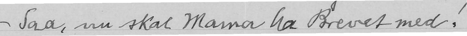- Saa, nu skal Mama ha Brevet med!
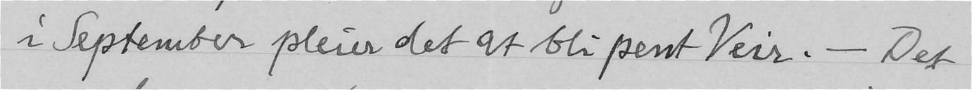i September pleier det at bli pent Veir. - Det
